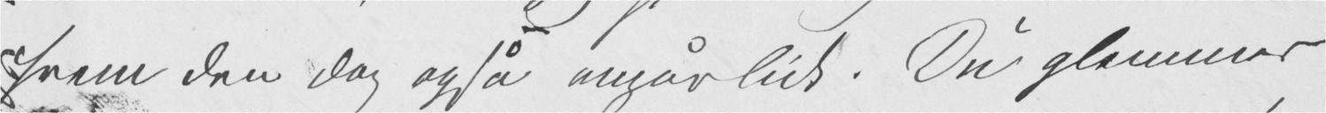hvem den dog også angår lidt. Du glemmer
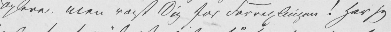opleve, men vogt Dig for Forvexlingen! Har jeg
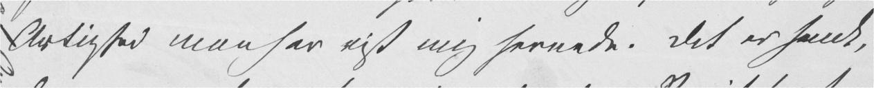Artighed man har vist mig hernede. Det er sandt,
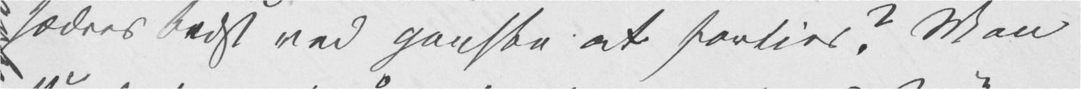hædres bedst ved ganske at forties? Man
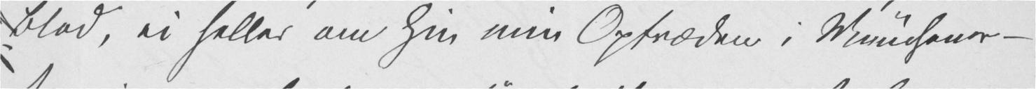Blad, ei heller om Hin min Optræden i Münchener-
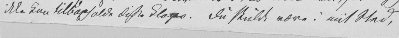ikke kan tilbageholde disse Klager. Du skulde være i mit Sted,
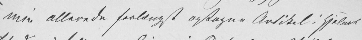min allerede forlængst optagne Artikel i Hjelms
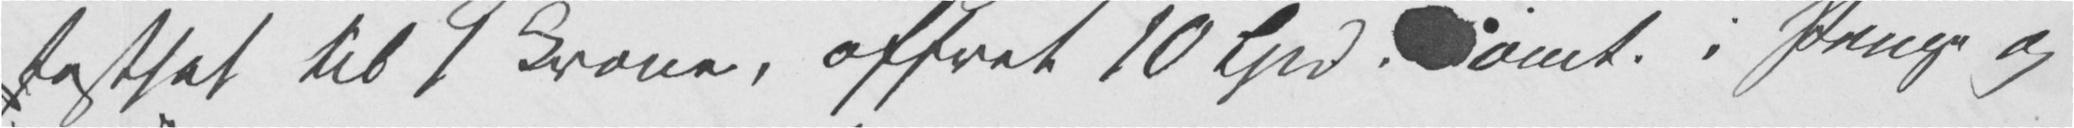fastsat til 1 Krone, offret 10 Spd omt. i Penge og
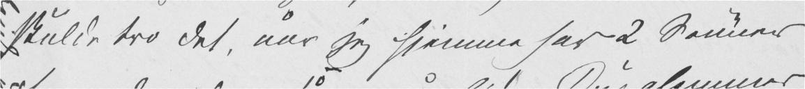skulde tro det, når jeg hjemme har 2 Sønner
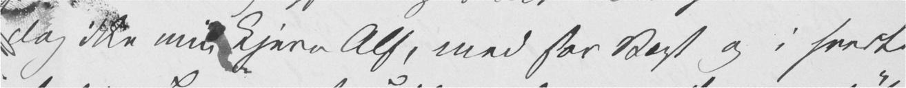dog ikke min kjere Alf, med stor Vægt og i hvert
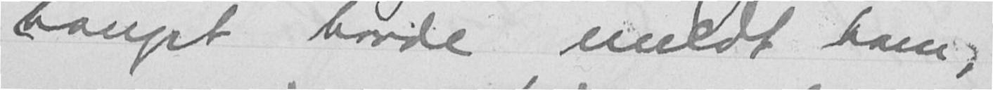haupt havde meldt ham,
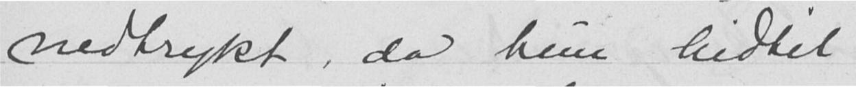nedtrykt, da hun hidtil
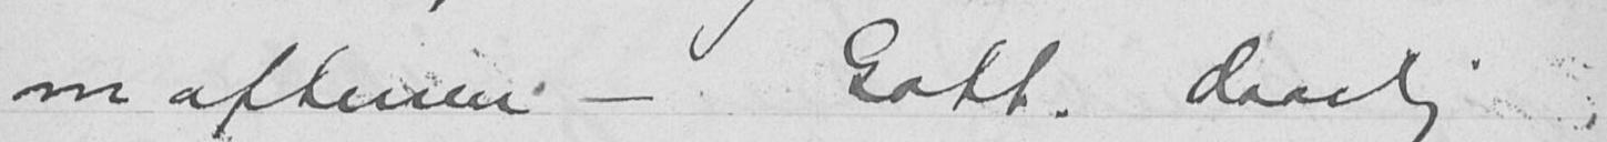om aftenen - Gott. daarlig
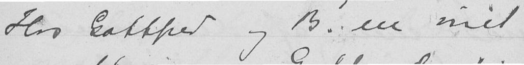Hos Gottfred og B. en visit
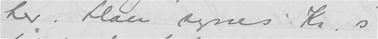her. Han synes Kr.s
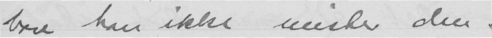bare han ikke mister den.
